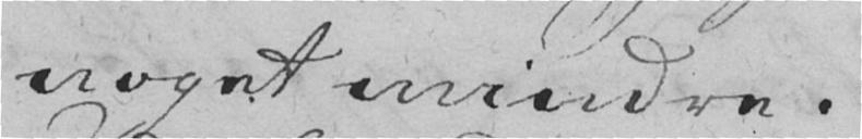noget mindre.
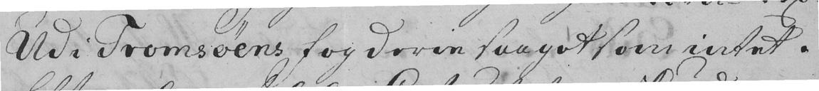Udi Tromsøens Fogderie saa got som intet.
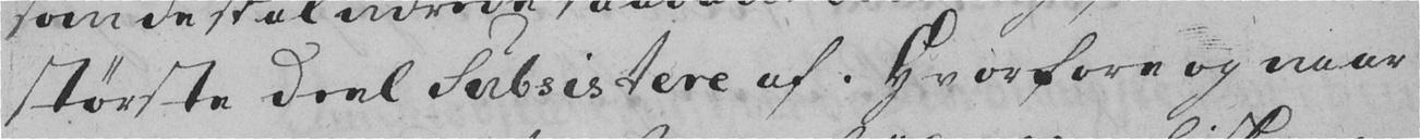største deel Subsistere af. Hvorfore og naar
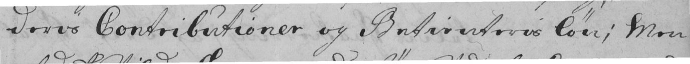deres Contributioner og Betienteris løn; Men
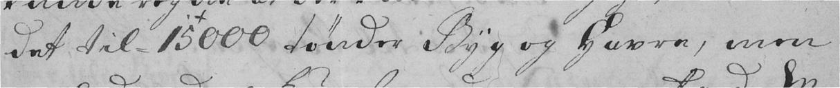det til 15000 tønder Byg og Havre, men
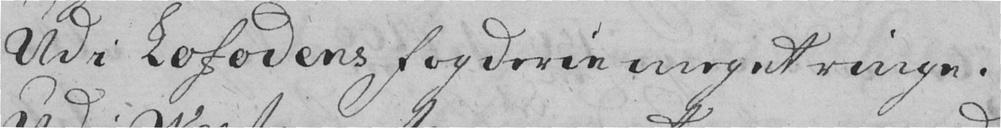Udi Lofodens Fogderie meget ringe.
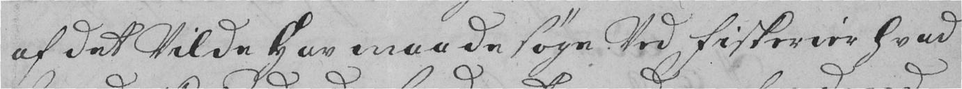af det Vilde Hav maa de søge ved fiskerier hvad
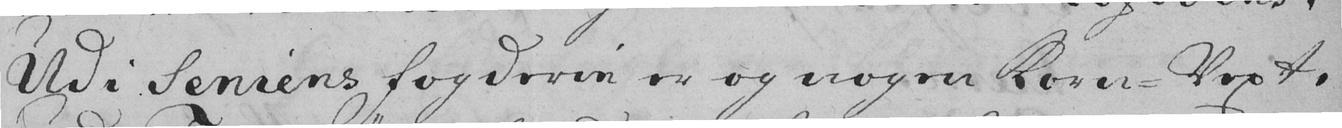Udi Seniens Fogderie er og nogen Korn-Vext.
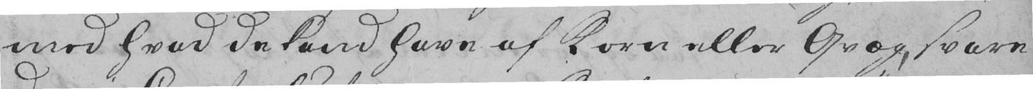med hvad de kand have af korn eller qvæg, svare
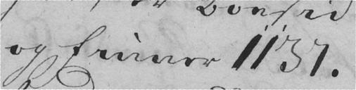og Finner 1137.
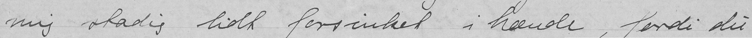mig stadig lidt forsinket i hænde, fordi du
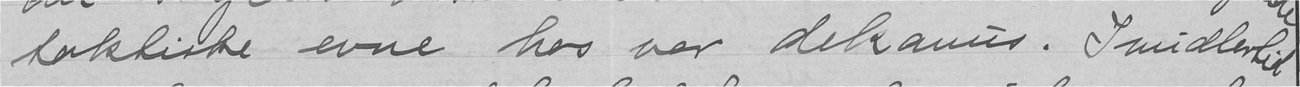taktiske evne hos vor dekanus. Imidlertid
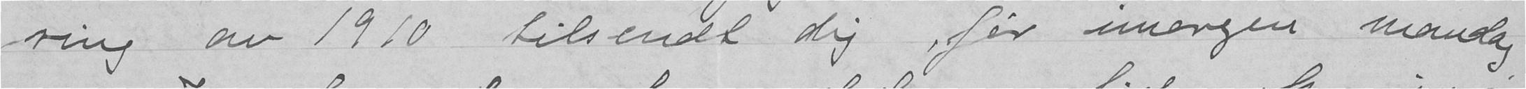ring av 1910 tilsendt dig før imorgen mandag
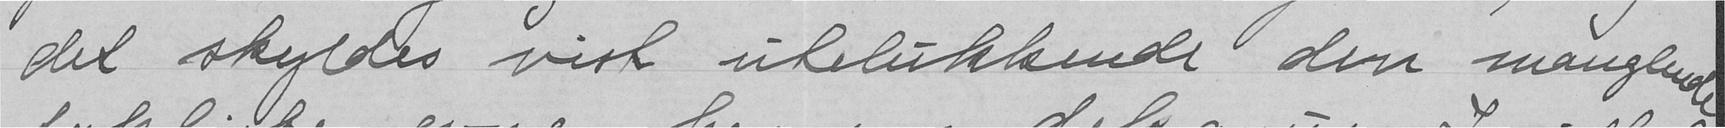det skyldes vist utelukkende den manglende
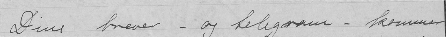Dine brever - og telegram - kommer
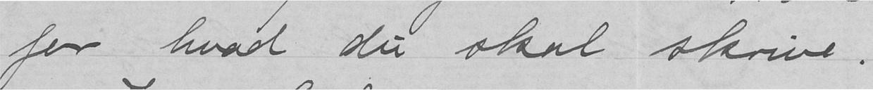for hvad du skal skrive.
